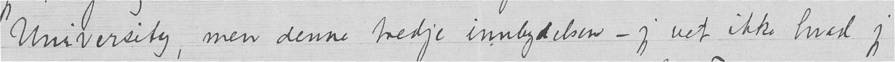University, men denne tredje innbydelsen – jeg vet ikke hvad jeg
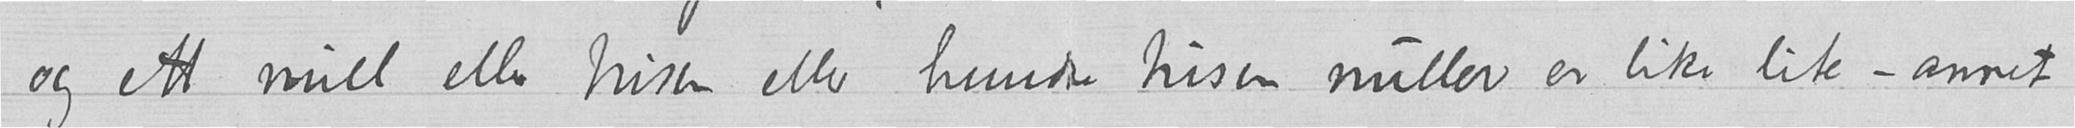og ett null eller tusen eller hundre tusen nuller er like lite – annet
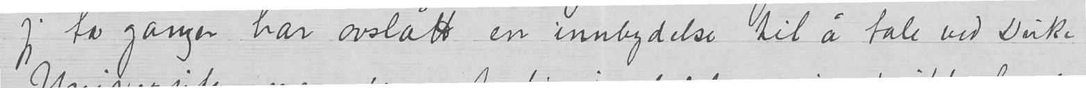jeg to gangen har avslått en innbydelse til å tale ved Duke
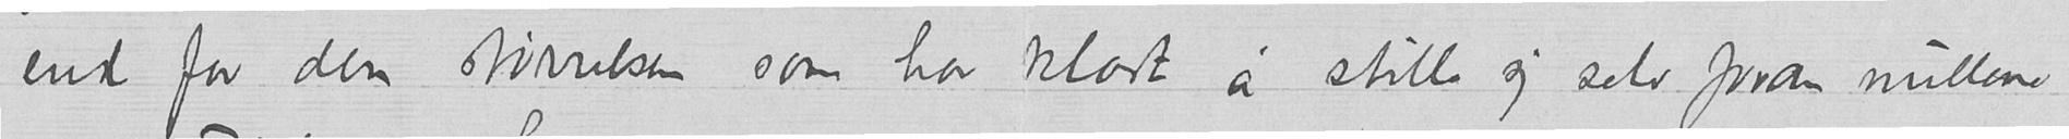end for den størrelsen som har klart å stille sig selv foram nullene
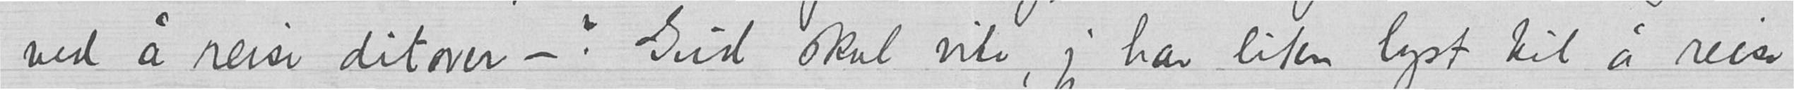ved å reise ditover – ? Gud skal vite, jeg har liten lyst til å reise
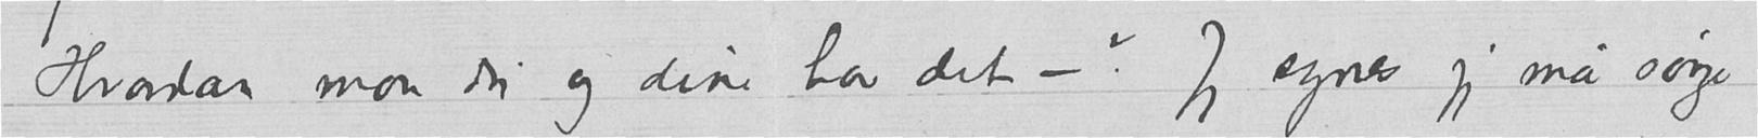Hvordan mon du og dine har det – ? Jeg synes jeg må sørge
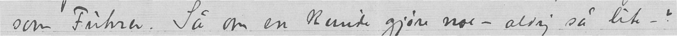som Fuhrer. Så om en kunde gjøre noe – aldrig så lite – ?
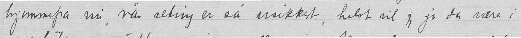hjemmefra nu, når alting er så usikkert, helst vil jeg jo da være i
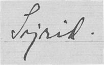Sigrid.
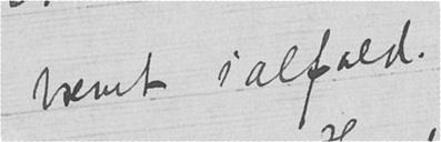brevet ialfald.
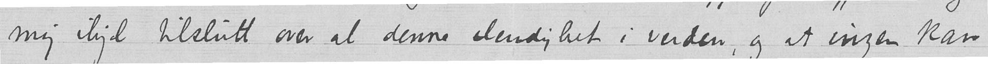mig ihjel tilslutt over al denne elendighet i verden, og at ingen kan
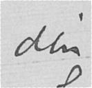din
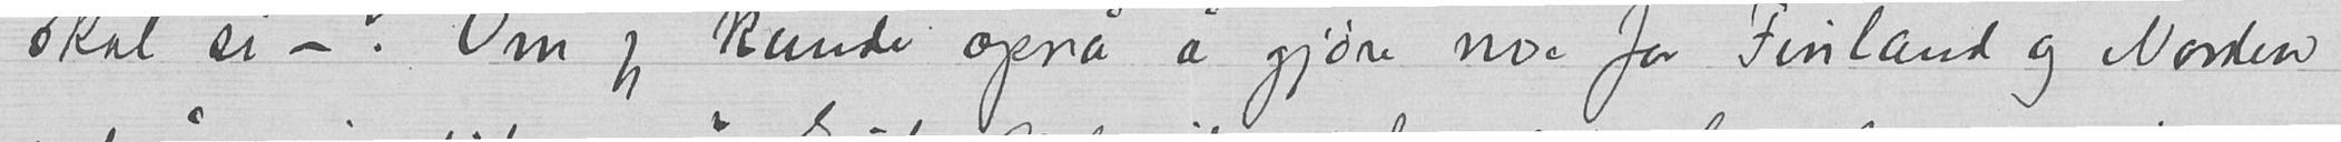skal si – ? Om jeg kunde opnå å gjøre noe for Finland og Norden
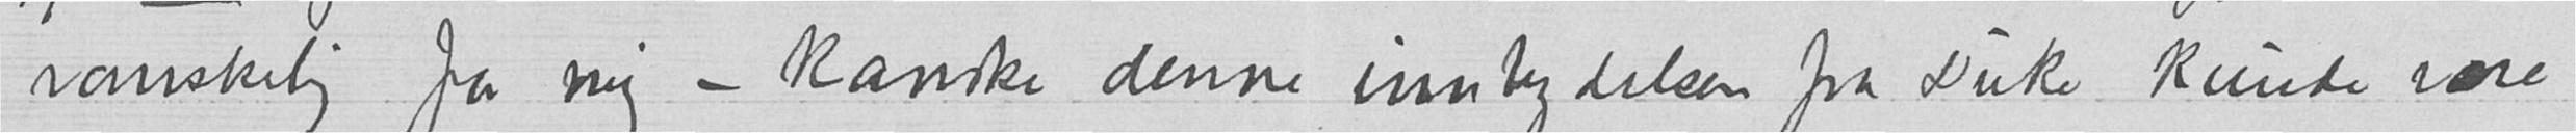vanskelig for mig – kanske denne innbydelsen fra Duke kunde være
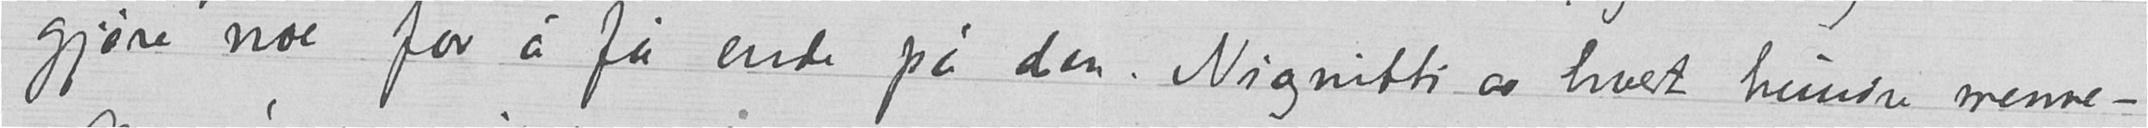gjøre noe for å få ende på den. Niognitti av hvert hundre menne –
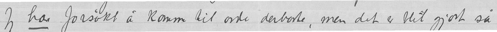Jeg har forsøkt å komme til orde derborte, men det er blit gjort så
